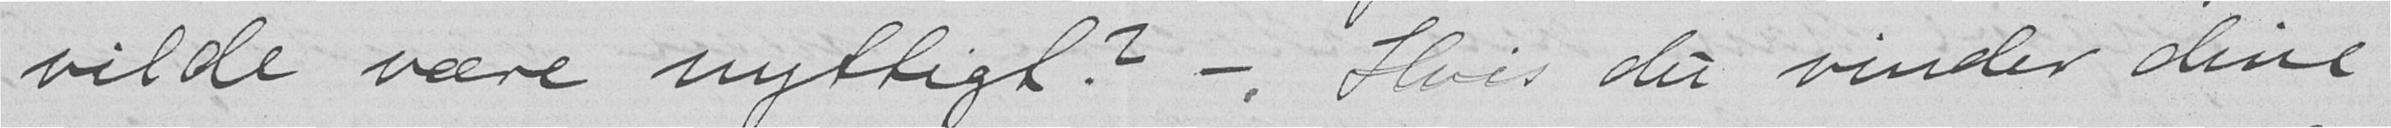vilde være nyttigt? – Hvis du vinder dine
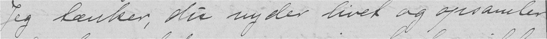Jeg tænker, du nyder livet og opsamler
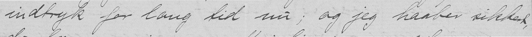indtryk for lang tid nu; og jeg haaber sikkert,
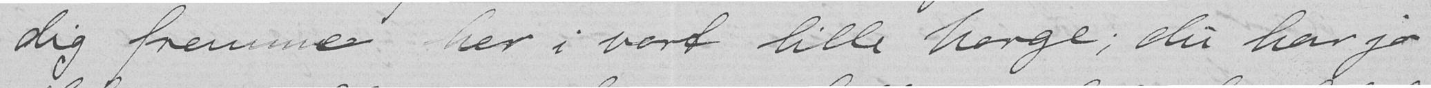dig fremme her i vort lille Norge; du har jo
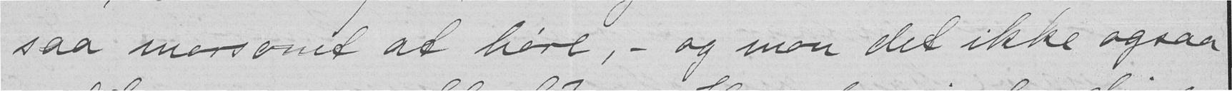saa morsomt at høre, – og mon det ikke ogsaa
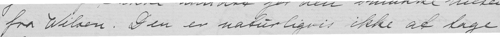fra Wilsen. Den er naturligvis ikke at tage
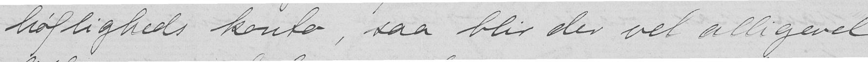høfligheds konto, saa blir der vel alligevel
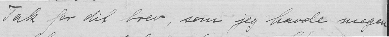Tak for dit brev, som jeg havde megen
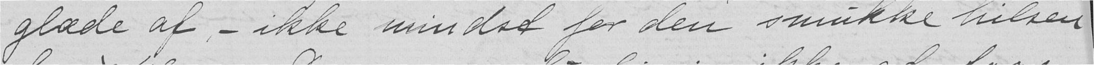glæde af, – ikke mindst for den smukke hilsen
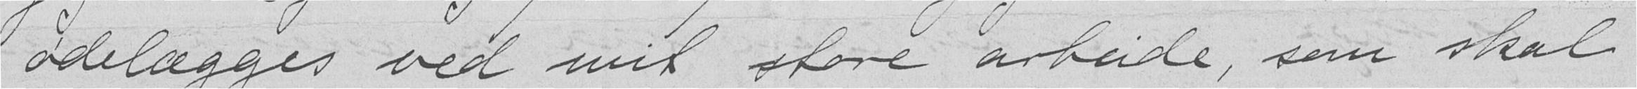ødelægges ved mit store arbeide, som skal
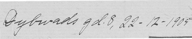Dybwads gd. 8, 22-12-1905
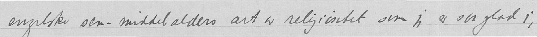engelske sen-middelalders art av religiøsitet som jeg er saa glad i,
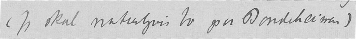(Jeg skal naturligvis bo paa Bondeheimen)
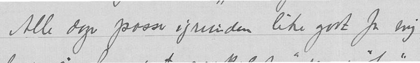Alle dager passer igrunden like godt for mig,
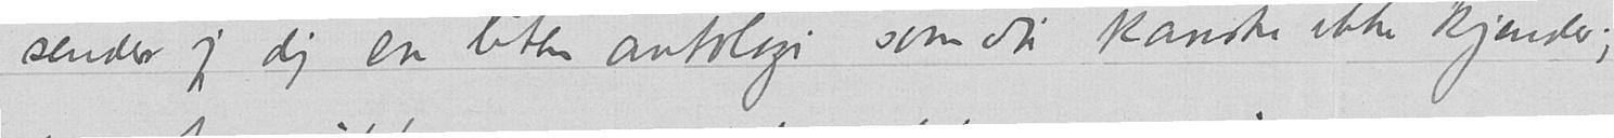sender jeg dig en liten antologi som du kanske ikke kjender;
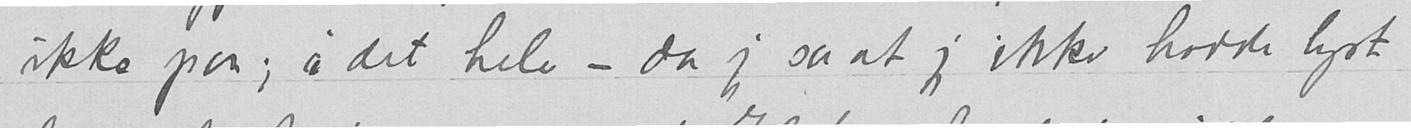ikke paa; i det hele - da jeg sa at jeg ikke hadde lyst
